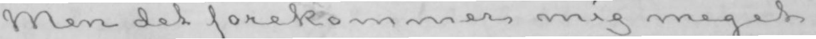Men det forekommer mig meget
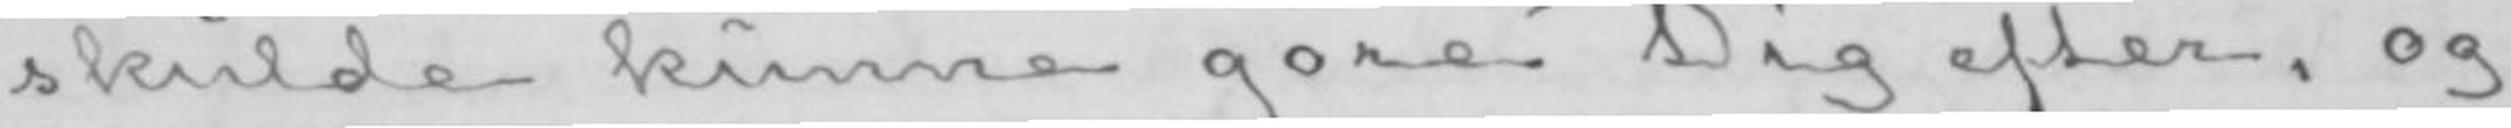skulde kunne gøre Dig efter, og
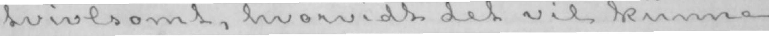tvivlsomt, hvorvidt det vil kunne
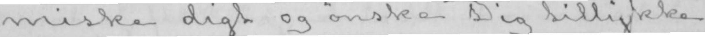miske digt og ønske Dig tillykke
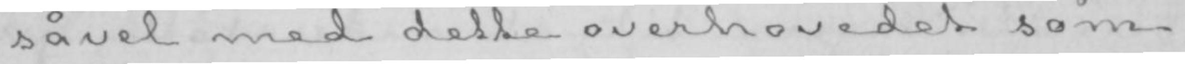såvel med dette overhovedet som
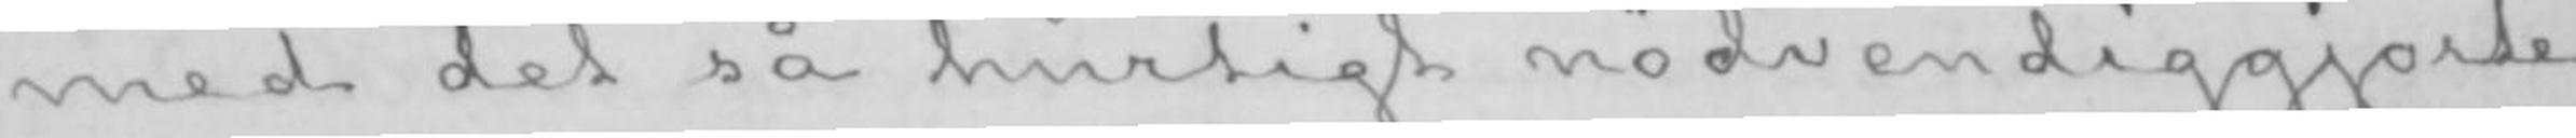med det så hurtigt nødvendiggjorte
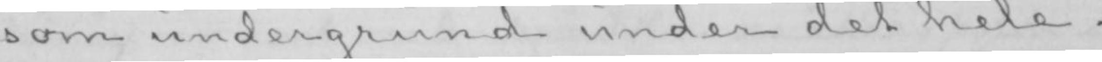som undergrund under det hele.
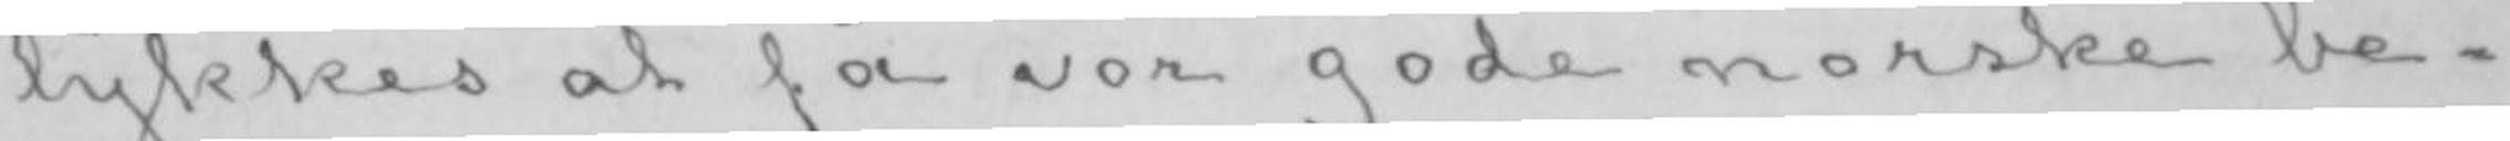lykkes at få vor gode norske be-
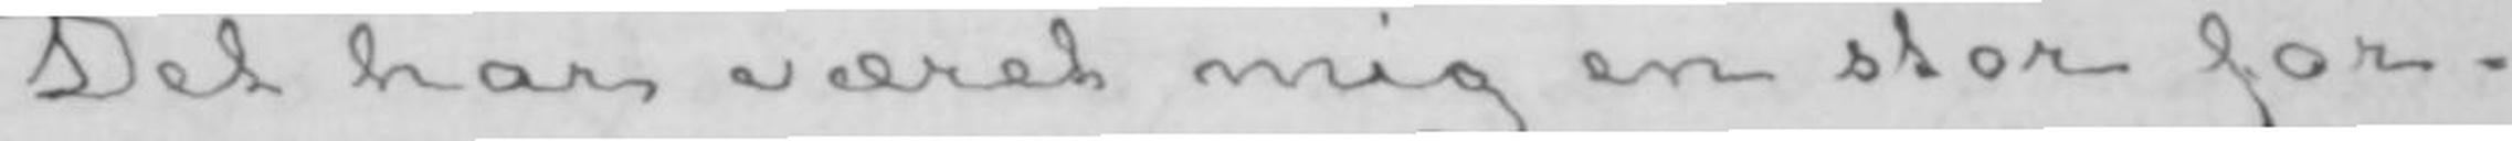Det har været mig en stor for-
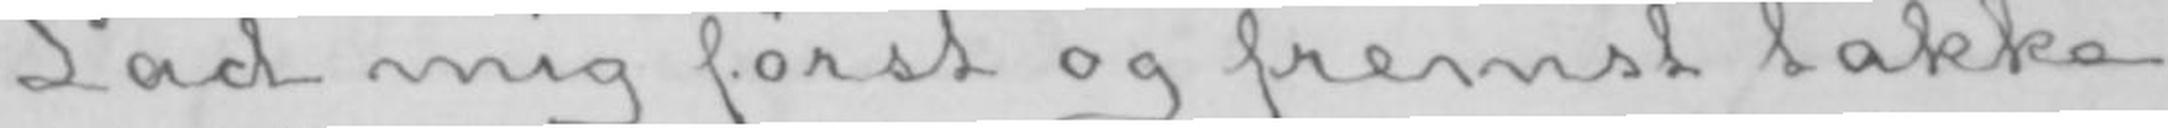Lad mig først og fremst takke
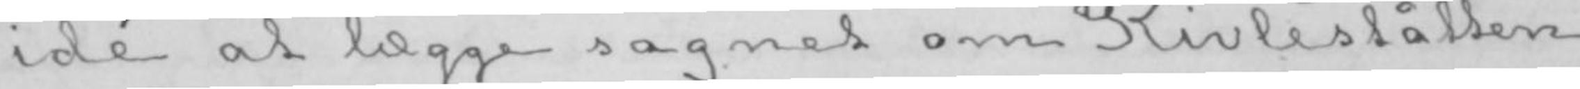idé at lægge sagnet om Kivleslåtten
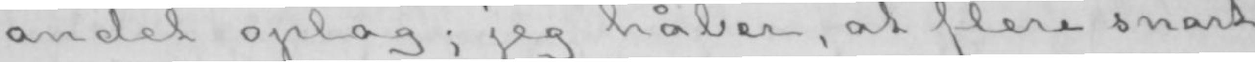andet oplag; jeg håber, at flere snart
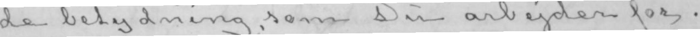de betydning, som Du arbejder for.
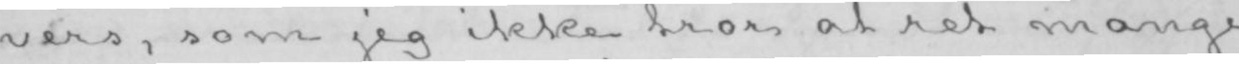vers, som jeg ikke tror at ret mange
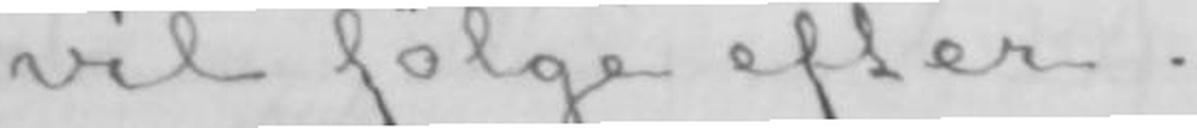vil følge efter.
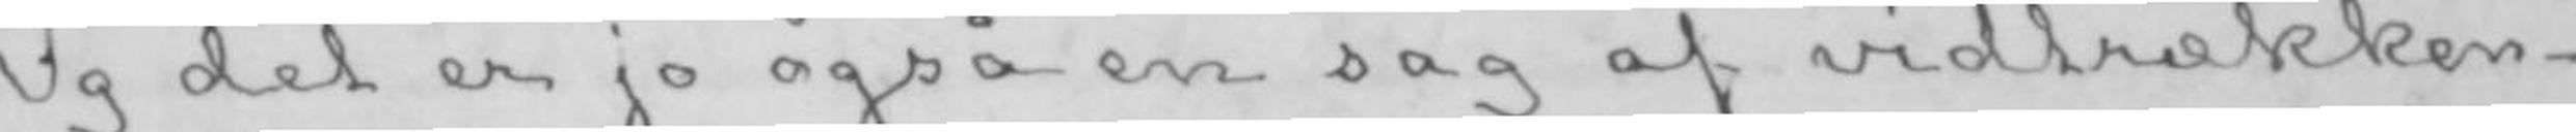Og det er jo også en sag af vidtrækken-
